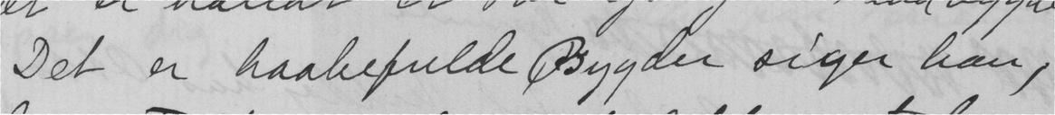Det er haabefulde Bygder siger han,
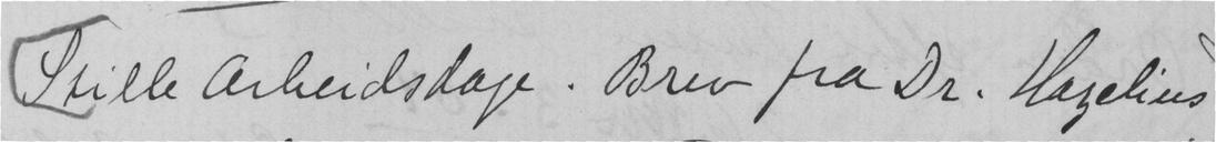Stille Arbeidsdage. Brev fra Dr. Hagelius
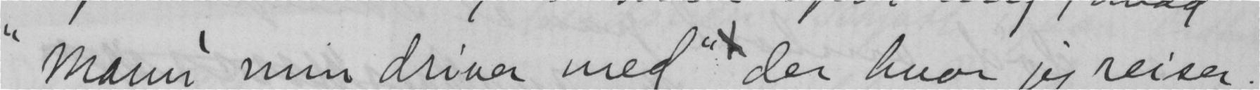"mann' min driver med" der hvor jeg reiser.
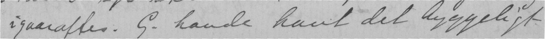igaaraftes. G. havde havt det hyggeligt
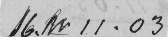16.  11.03
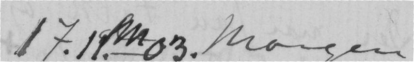17.11.03. morgen
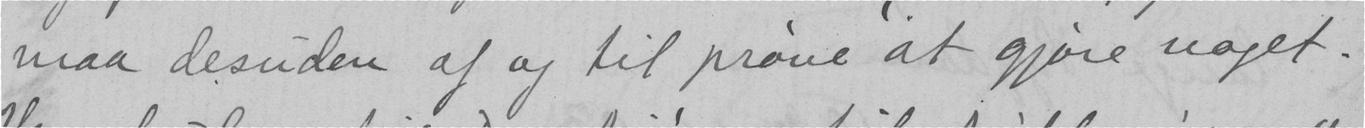maa desuden af og til prøve at gjøre noget.
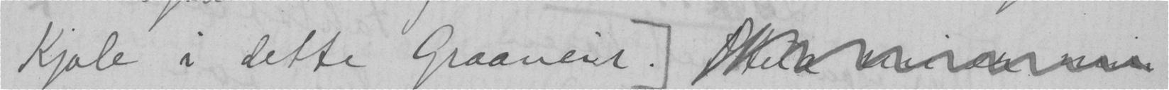Kjole i dette Graaveir.
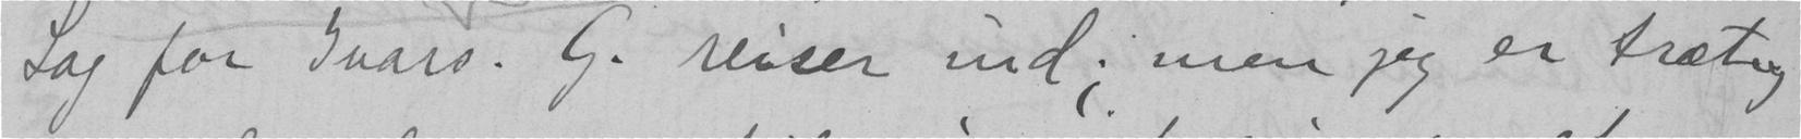Lag for Ivars. G. reiser ind; men jeg er træt og
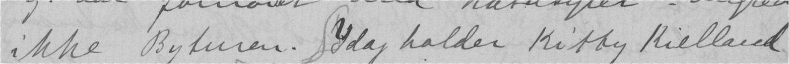ikke Bytuen. Idag holder Kitty Kielland
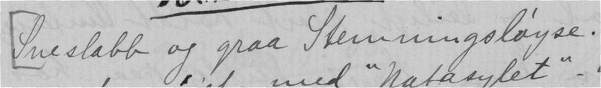Sneslabb og graa Stemningsløyse.
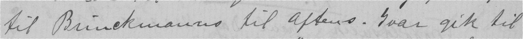til Brinckmanns til aftens. Ivar gik til
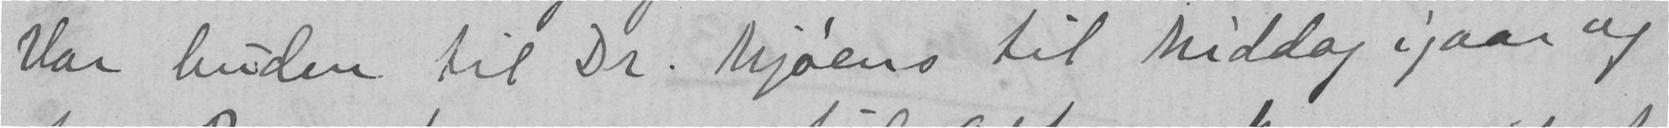Var buden til Dr. Mjøens til Middag igaar og
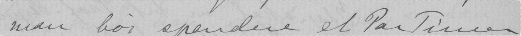man bør spandere et Par Timer
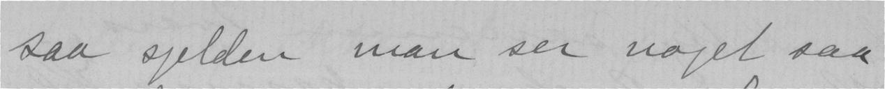saa sjelden man ser noget saa
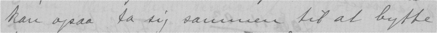kan ogsaa ta sig sammen til at bytte
